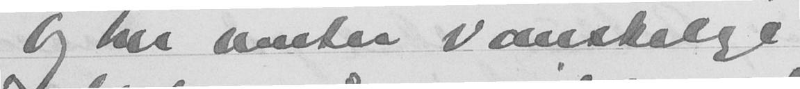Og her venter vanskelige
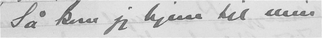Så kom jeg hjem til min
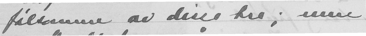følsomme av disse tre; men
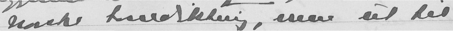norske folkedikting, men ut til
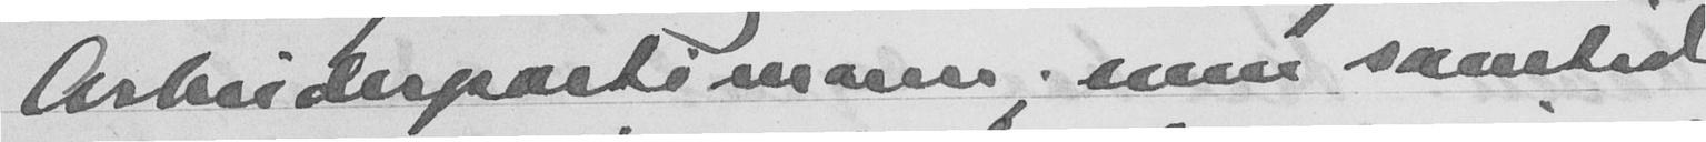Arbeiderpartimann, men samtidig
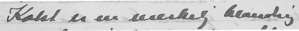Koht er en merkelig blanding
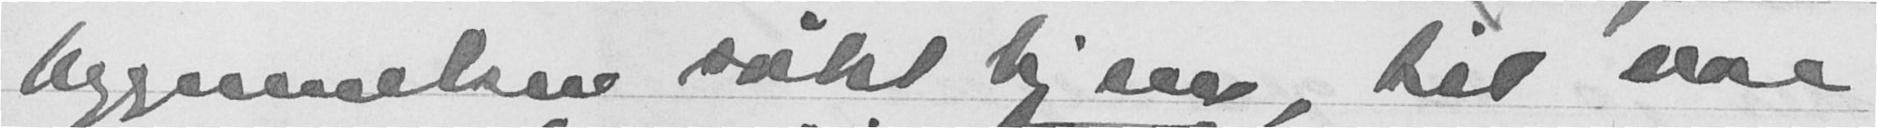bygemelsen vårt hjem, til sæe
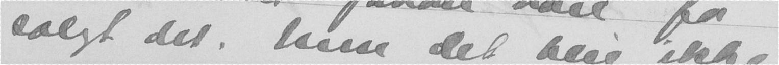solgt det. Men det blir ikke
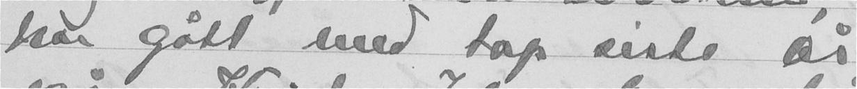her gått med tap siste år
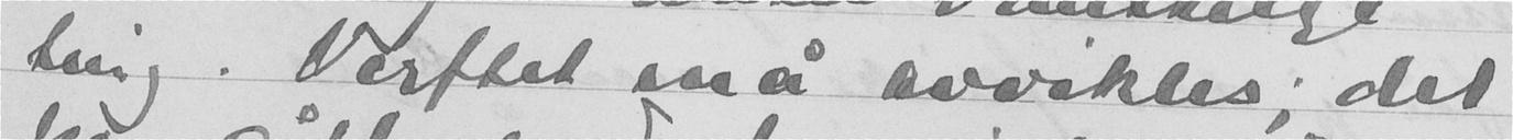ting. Verftet må avvikles; det
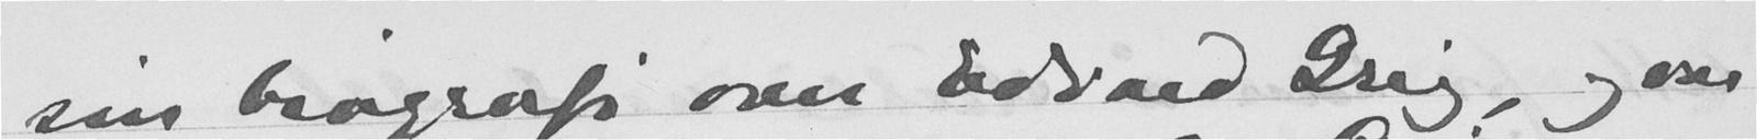sin brografi over Edvard Grieg, og var
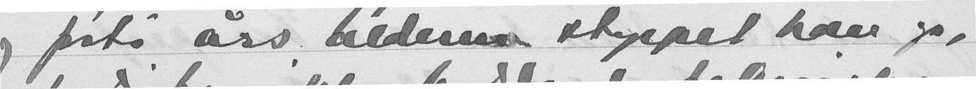og fårstå års bedere stippet kan op,
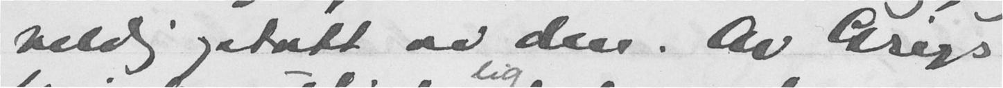veldig optatt av den. Av Griegs
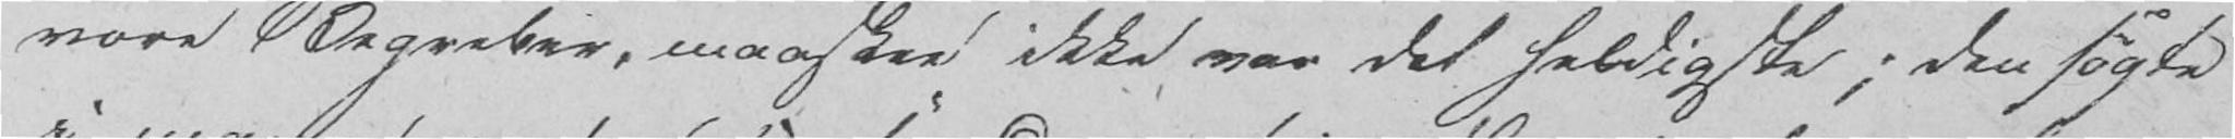vore Begreber, maaskee ikke var det heldigste; den søgte
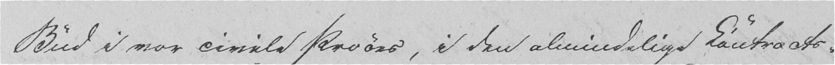Bud i vor civile Proces, i den almindelige Kontracts-
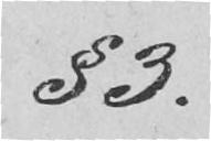§ 3.
